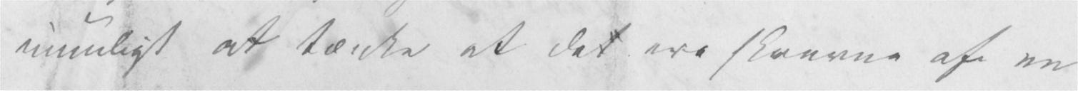umuligt at tænke at det ere skrevne af en
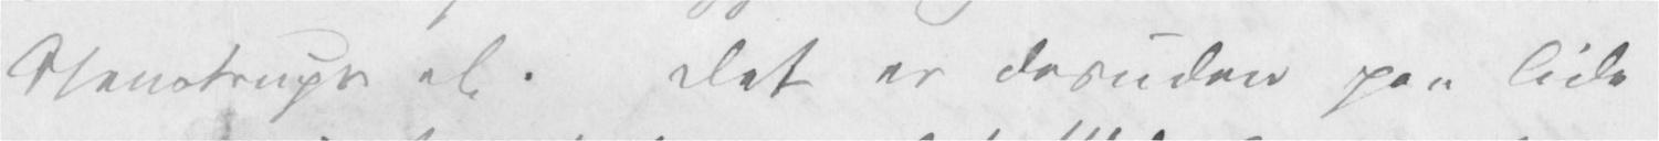Stenstrups etc. Det er desuden paa Tide
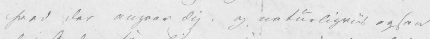hvad der angaar Dig, og naturligviis ogsaa
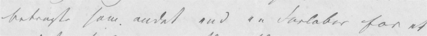betragte som andet end en Forløber for et
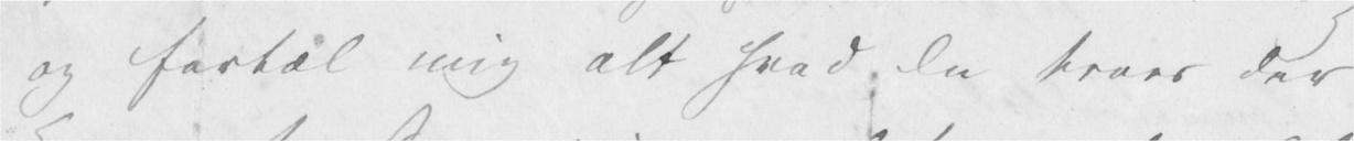og fortæl mig alt hvad Du troer der
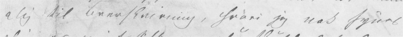Dig til Brevskrivning, hvori jeg nok synes
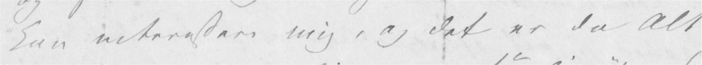kan interessere mig, og det er da Alt
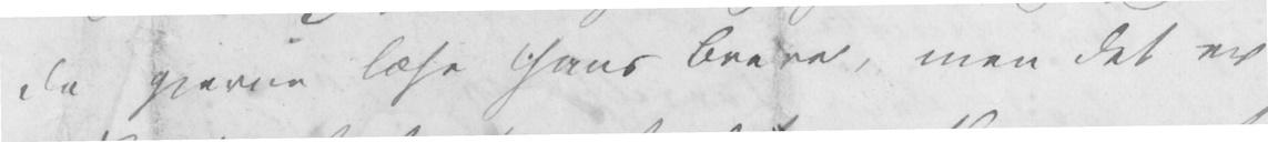da gjerne læse hans Breve, men det er
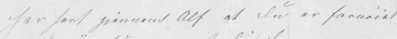har havt gjennem Alf at Du er fornøiet
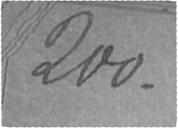200.
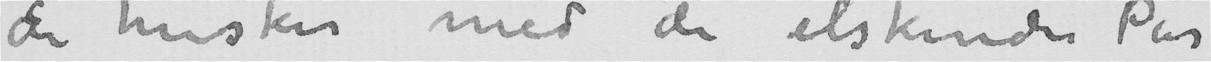De husker med de elskende Par
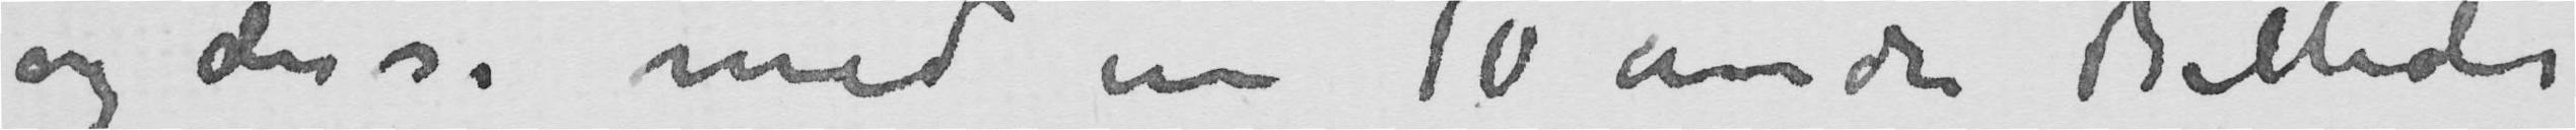og disse med en 10 andre Billeder
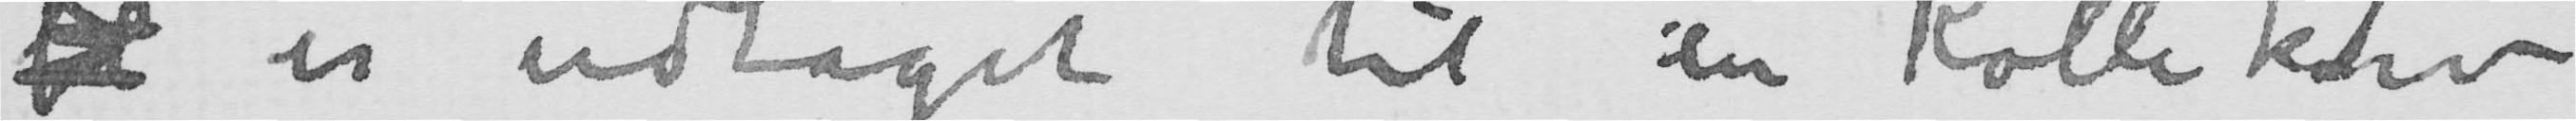er udtaget til en Kollektiv
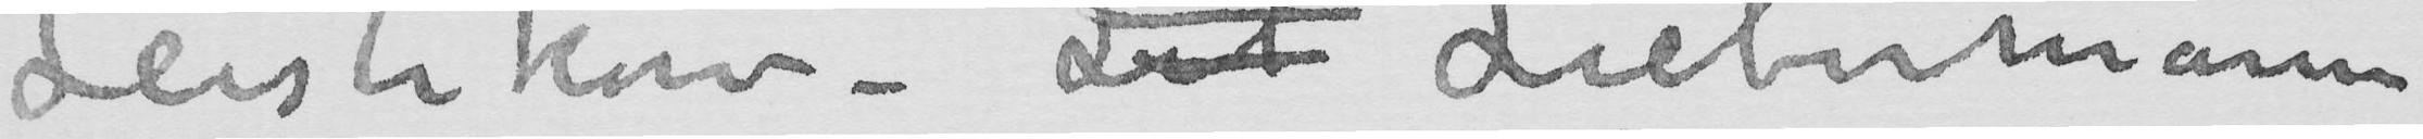Leistikow – Lib Liebermann
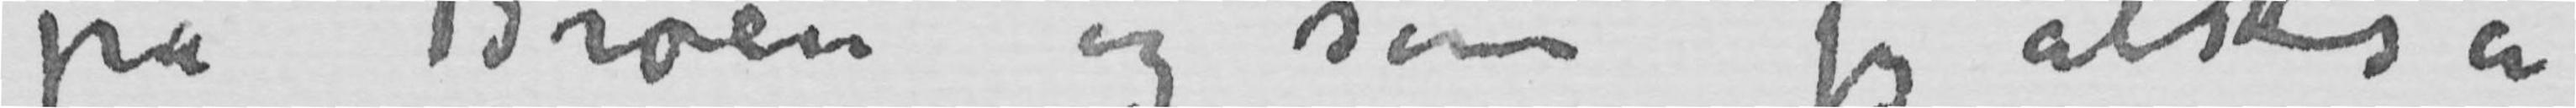på Broen og som jeg altså
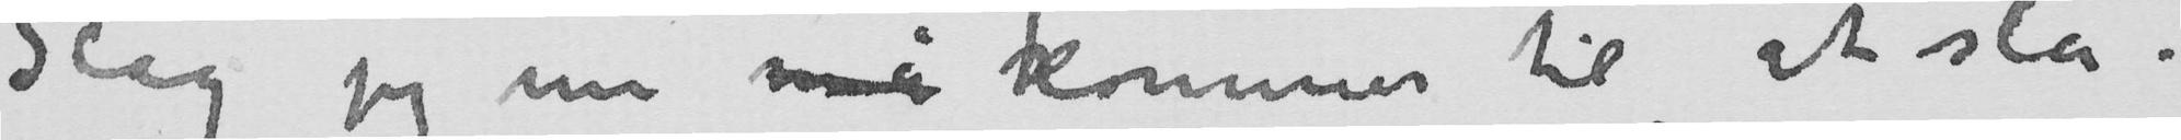Slag jeg nu må kommer til at slå
